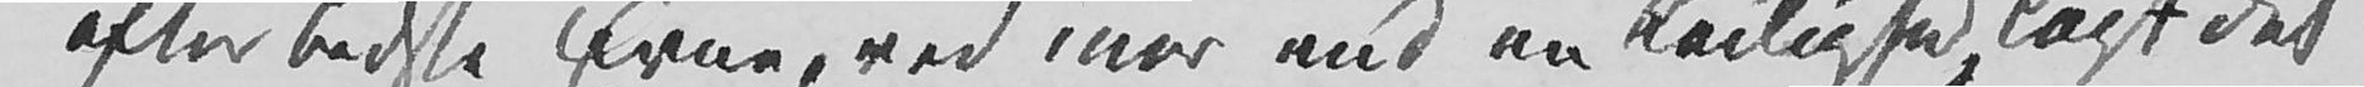efter bedste Evne, ved mer end en Leilighed, lagt det
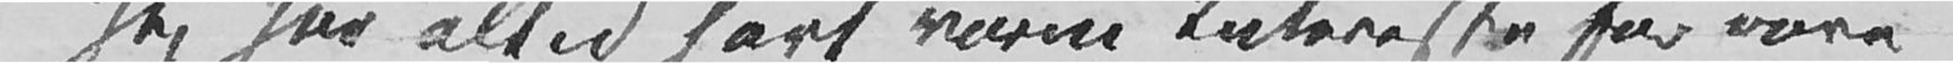Jeg har altid havt varm Interesse for vore
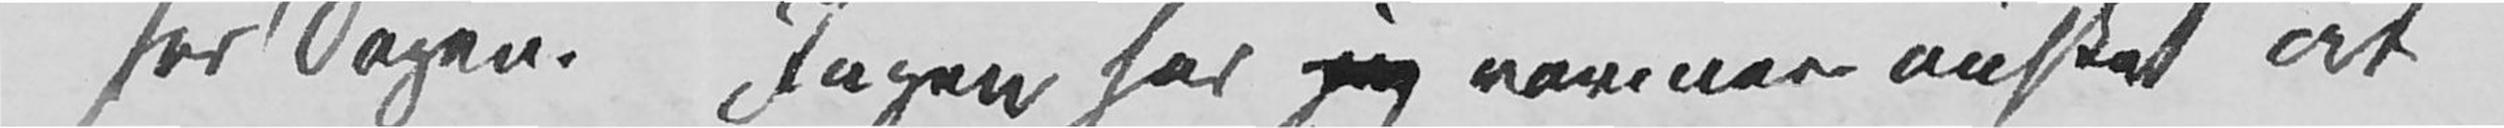for Dagen. Ingen har jeg varmere ønsket at
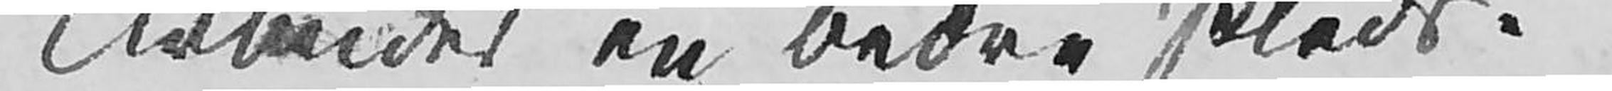Arbeider en bedre Plads.
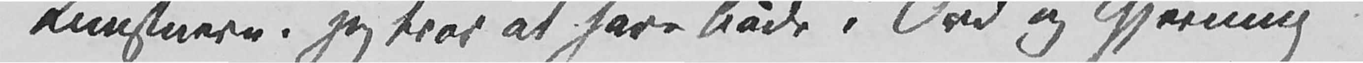Kunstnere. Jeg tror at have både i Ord og Gjerning
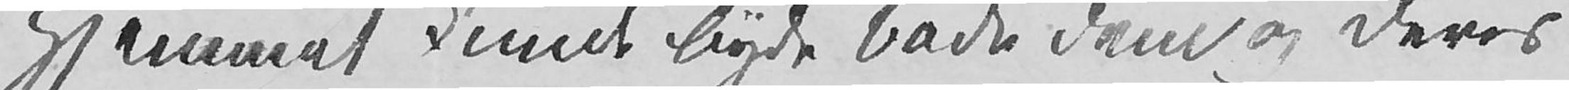Hjemmet kunde byde både dem og deres
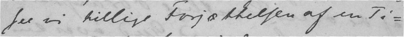see vi tillige Forjættelsen af en Ti-
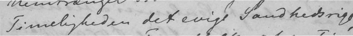Timeligheden det evige Sandhedsrige,
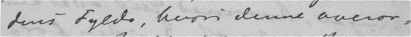dens Fylde, hvori denne overor-
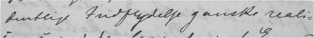dentlige Indflydelse ganske reali-
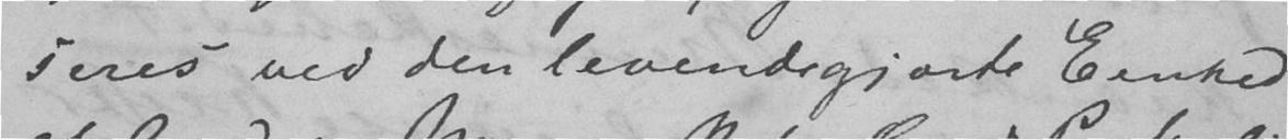seres ved den levendegjorte Eenhed
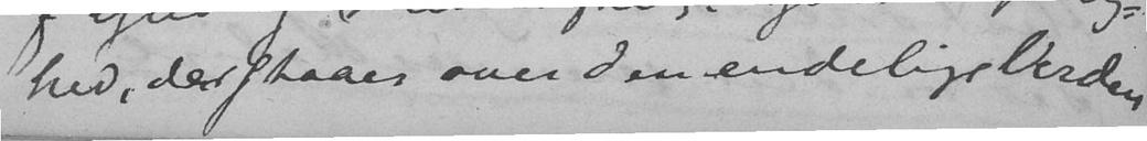hed, der staaer over den endelige Verden
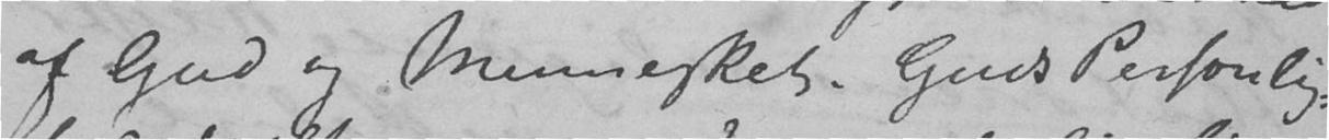af Gud og Mennesket. Guds Personlig-
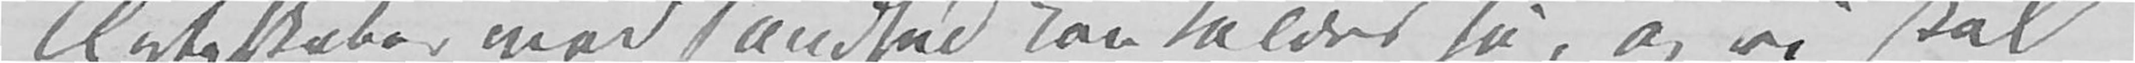Ægteskaber med Sandhed kan kaldes så, og vi skal
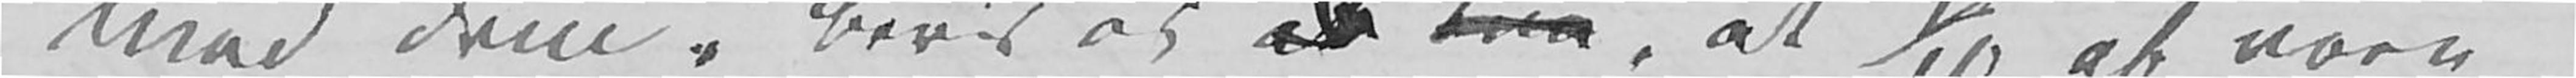med dem! bevis os at kun, at 9/10 af vore
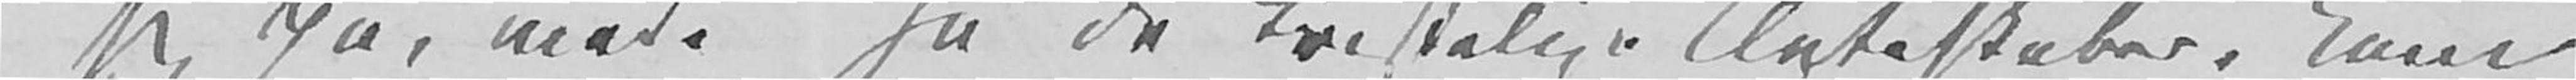sig på, med. Ja de «Kristelige Ægteskaber[»]. Kom
